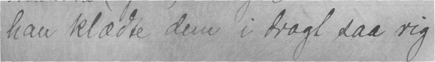han klædte dem i dragt saa rig
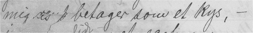mig  betager som et kys, -
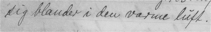sig blander i den varme luft.
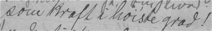som kraft i høiste grad!
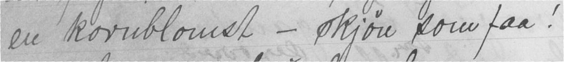en kornblomst - skjøn som faa!
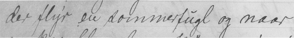der flyr en sommerfugl og naar
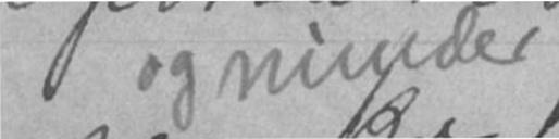og minder
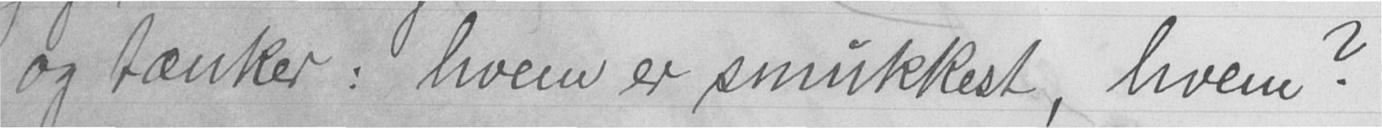og tænker: hvem er smukkest, hvem?
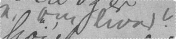om hvad?
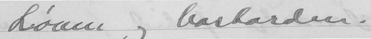Røver og bastarden,
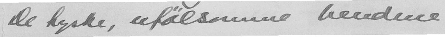De tyske, ufølsomme hendene!
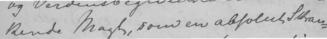kende Magt, som en absolut Skran-
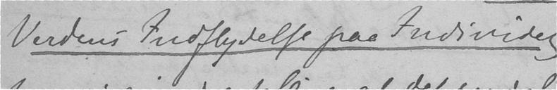Verdens Indflydelse paa Individet
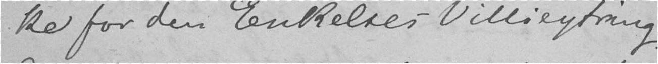ke for den Enkeltes Villiesytring
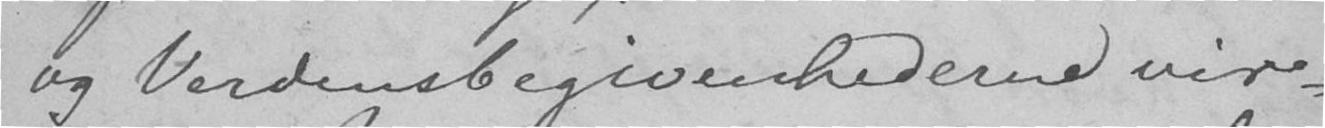og Verdensbegivenhederne vir-
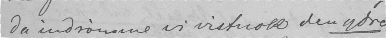da indrømme vi vistnok den ydre
